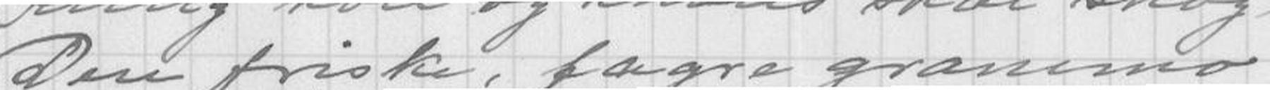Den friske, fagre grannemo
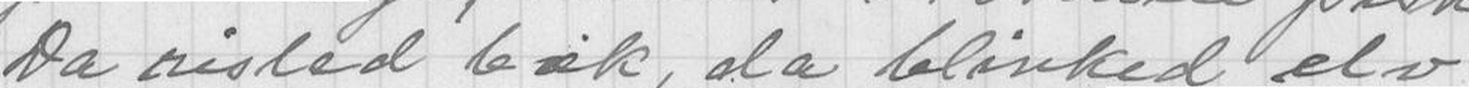Da risled bæk, da blinked elv
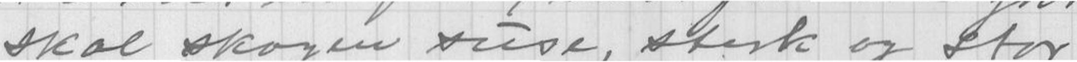skal skogen suse, sterk og stor
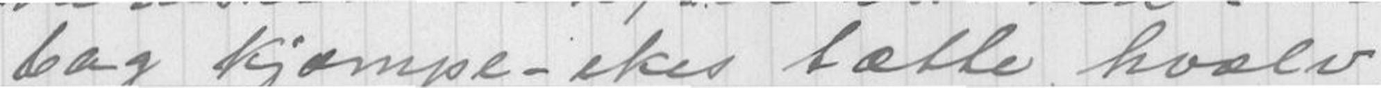bag kjæmpe - eker tætte hvælv,
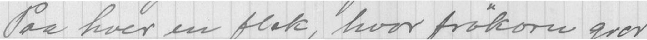Paa hver en flok, hvor frøkorn gror
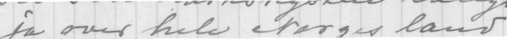ja over hele Norges land
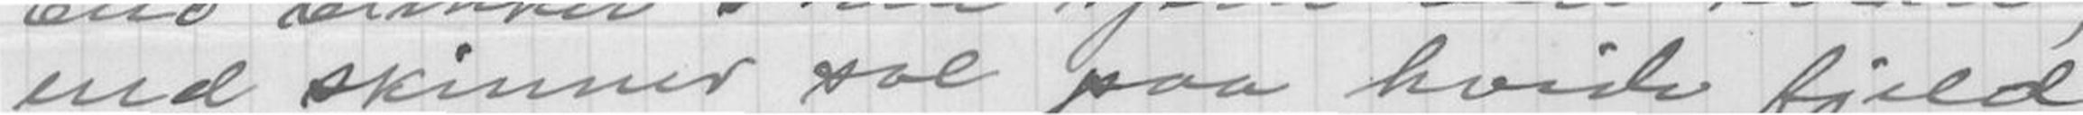med skimmer saa paa hvide fjeld
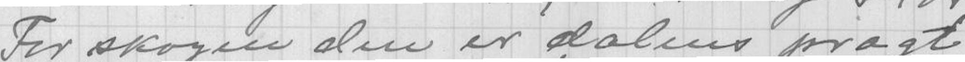For skogen den er dalens prakt
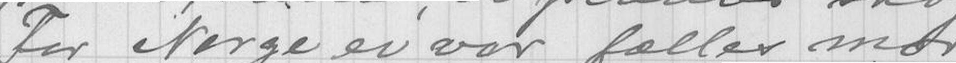For Norge er vor fælles mor
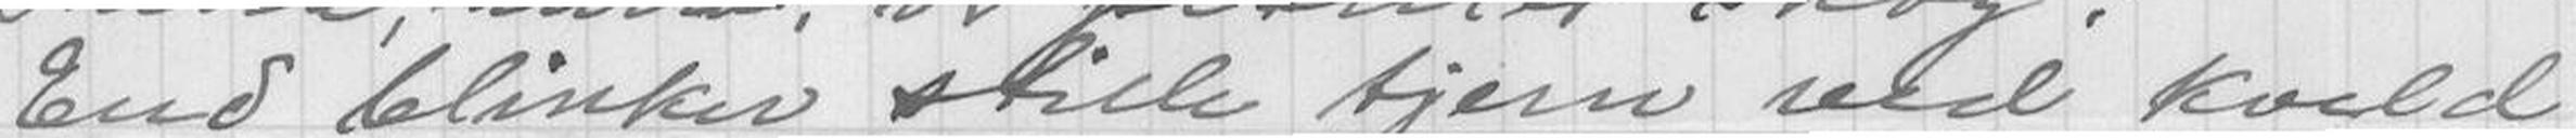End blinker stille tjern ved kveld
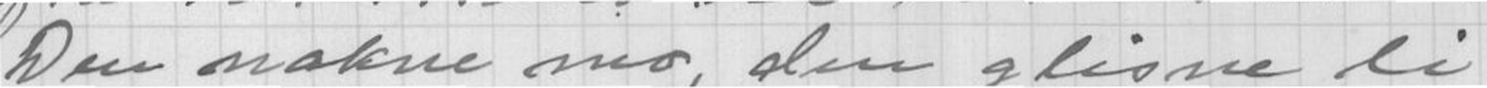Den nakne mo, den glisne li,
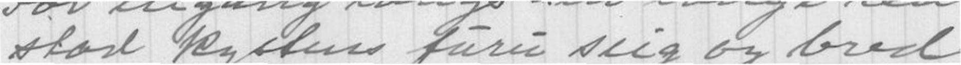stod Kystens furu slig og bred
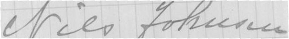Nils Johnsen
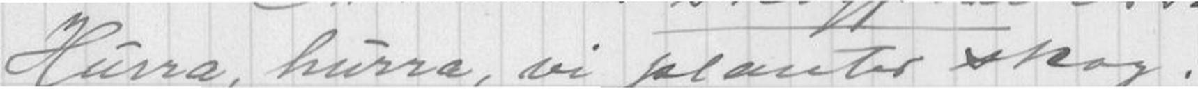Hurra, hurra, vi planter skog!
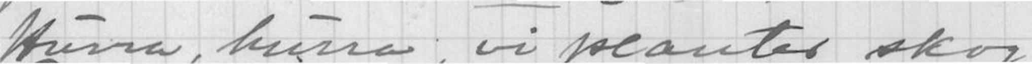Hurra, hurra, vi planter skog
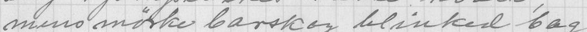mens mørke barskog blinked bag
